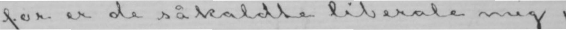for er de såkaldte liberale mig på
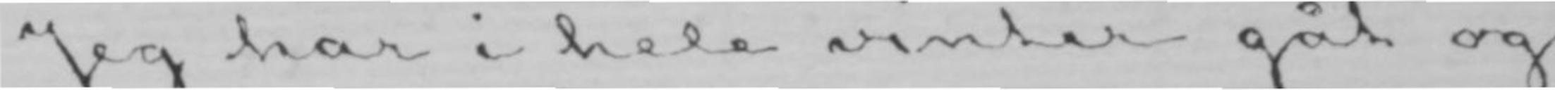Jeg har i hele vinter gåt og
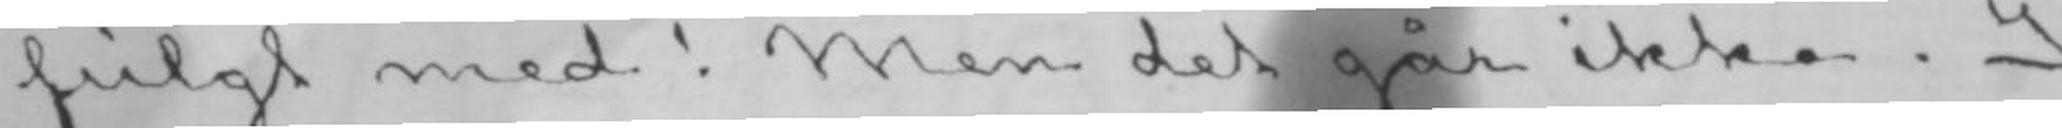fulgt med! Men det går ikke. I
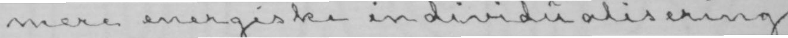mere energiske individualisering
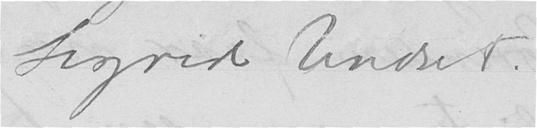Sigrid Undset.
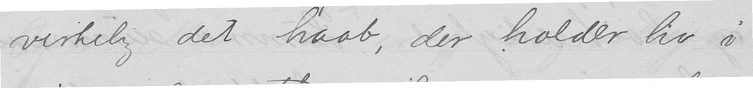virkelig det haab, der holder liv i
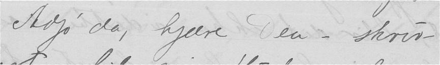Adjø da, kjære Dea - skriv
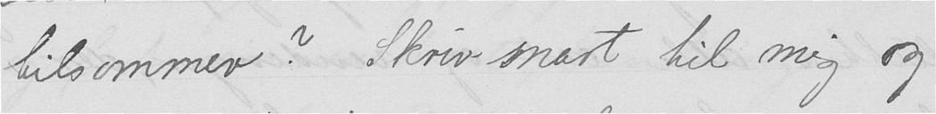tilsommer? Skriv snart til mig og
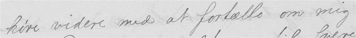køre videre med at fortælle om mig
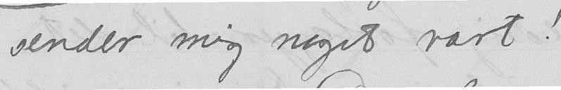mig noget snart!
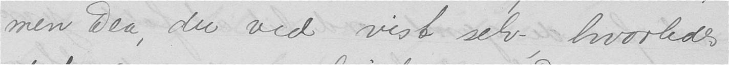men Dea, du ved vist selv, hvorledes
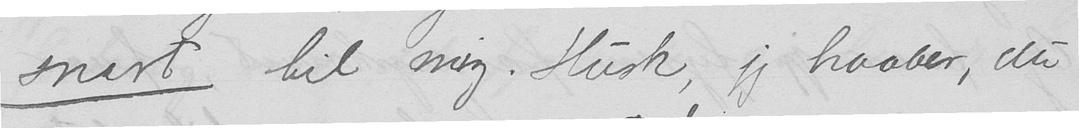snart til mig. Husk, jeg haaber, du sender
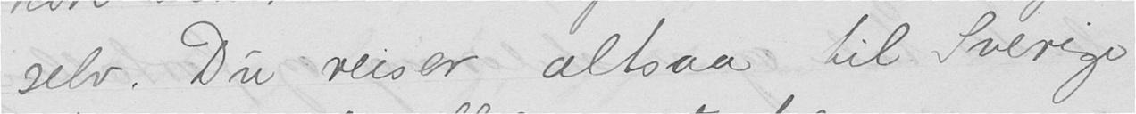selv. Du reiser altsaa til Sverige
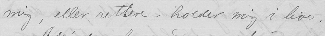mig, eller rettere - holder mig i live.
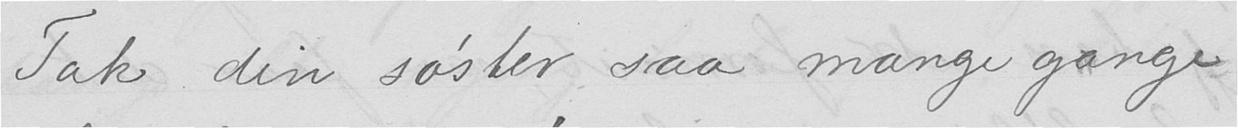Tak din søster saa mange gange
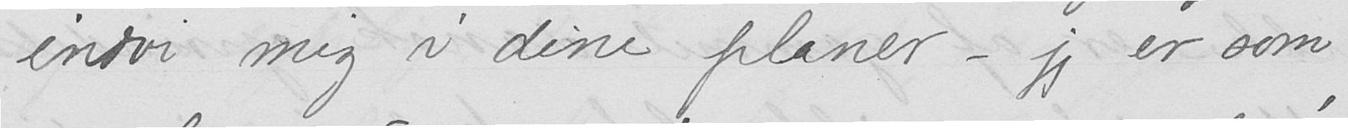indvi mig i dine planer - jeg er som
